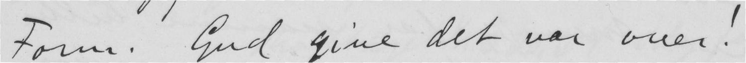Form. Gud give det var over!
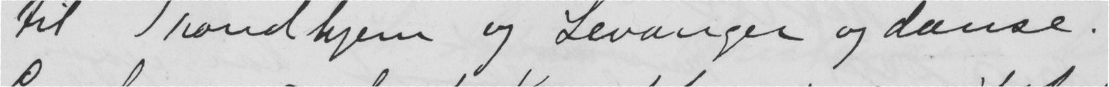til Trondhjem og Levanger og danse.
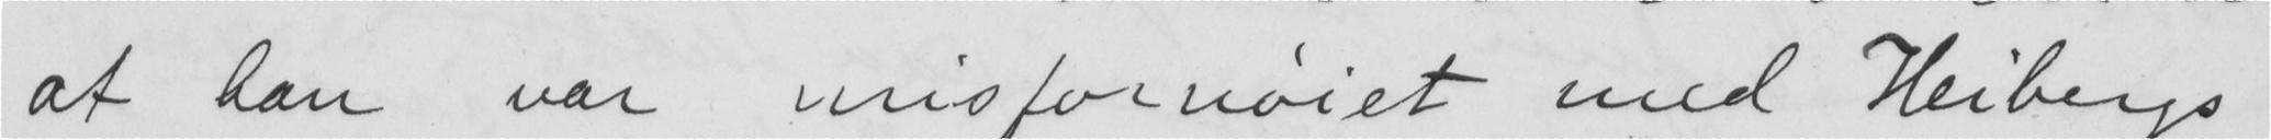at han var misfornøiet med Heibergs
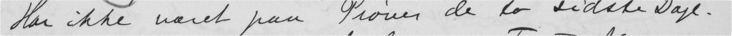Har ikke været paa Prøver de to sidste Dage.
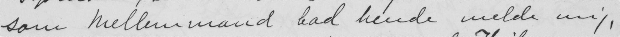som Mellemmand bad hende velde mig,
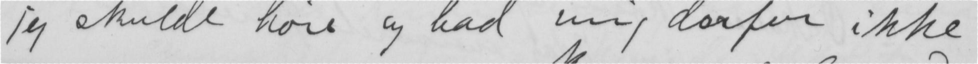Jeg skulde høre og bad mig derfor ikke
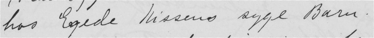hos Egede Nissens syge Barn.
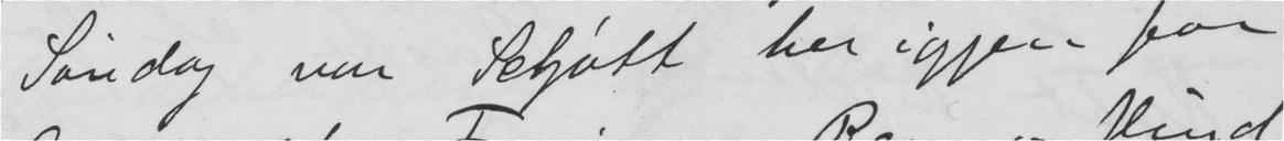Søndag var Schjøtt her igjen for
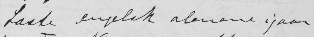Læste engelsk alenene igaar
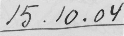15.10.04
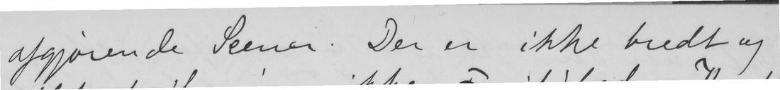afgjørende Scener. Der er ikke bredt og
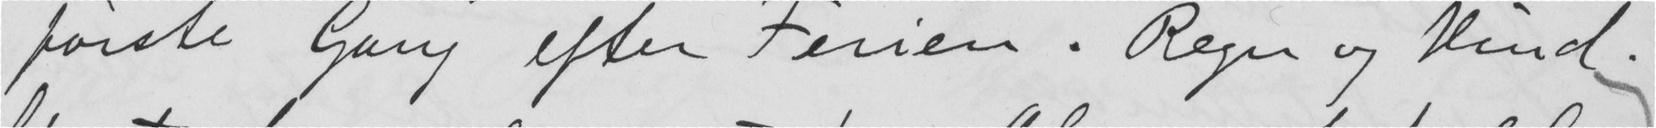første Gang efter Ferien. Regn og Vind.
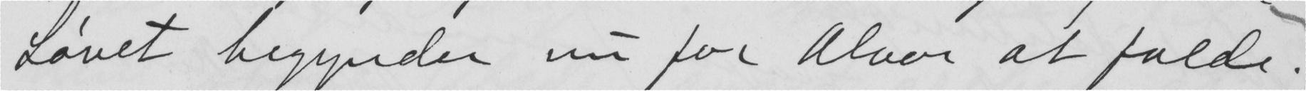Løvet begynder nu for Alvor at falde.
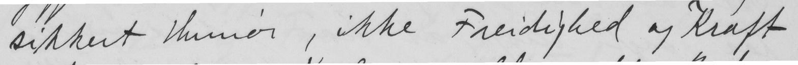sikkert Humør, ikke Freidighed og Kraft
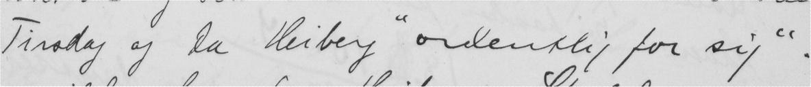Tirsdag og ta Heiberg "ordentlig for sig".
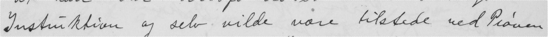Instruktion og selv vilde være tilstede ved Prøven
